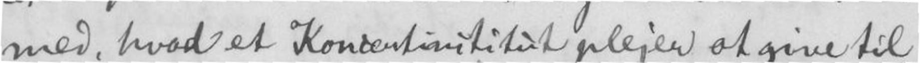med, hvad et Koncertinstitut plejer at give til
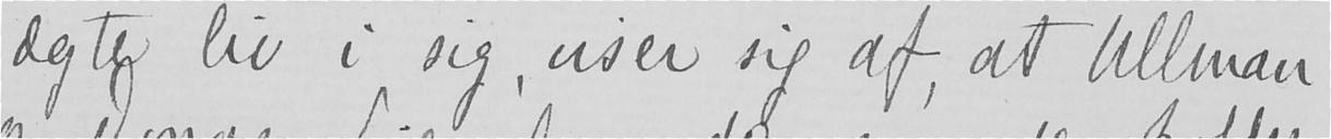ægte liv i sig, viser sig af, at Ullman
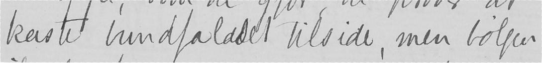kaste bundfaldet tilside, men bølgen
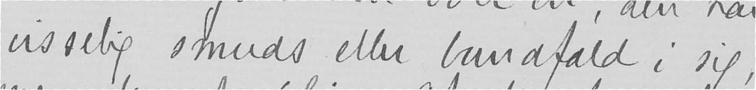visselig smuds eller bundfald i sig,
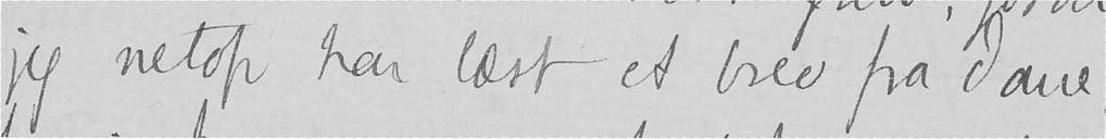jeg netop har læst et brev fra Jane,
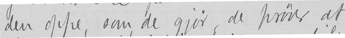den oppe, som de gjør de prøver at
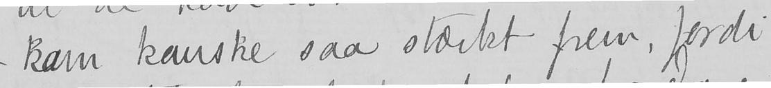kom kanske saa stærkt frem, fordi
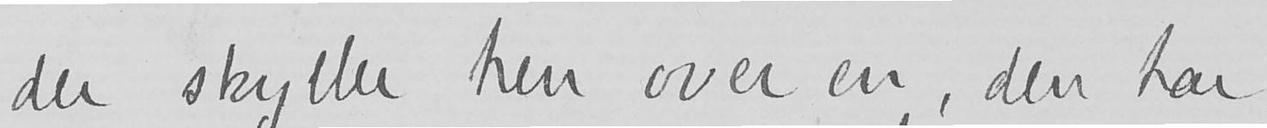der skyller hen over en, den har
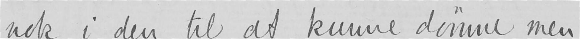nok i den til at kunne dømme, men
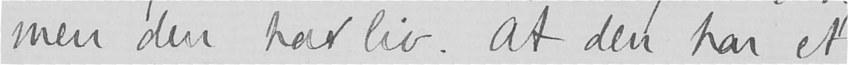men den har liv. At den har et
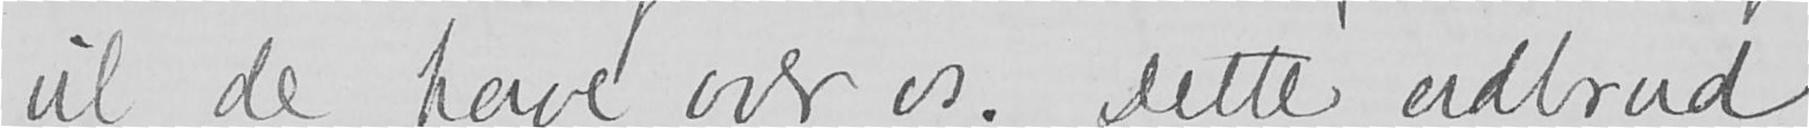vil de have over os. Dette udbrud
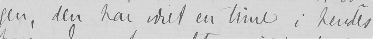gen, den har været en time i hendes
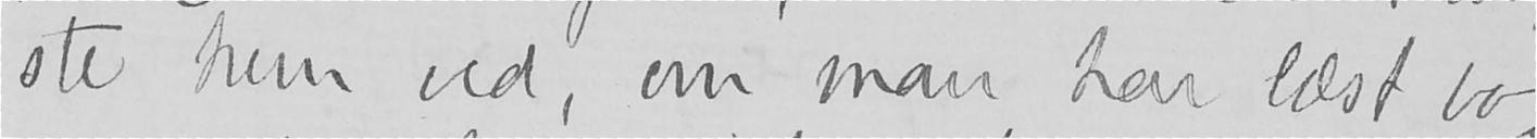ste hun ved, om man har læst bo-
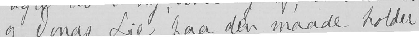og Jonas Lie paa den maade holder
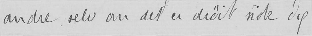andre selv om det er drøit nok. Jeg
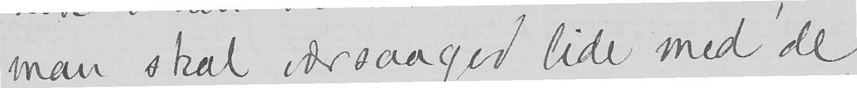man skal værsaagod lide med de,
